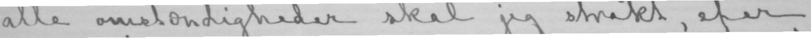alle omstændigheder skal jeg strakt, efer
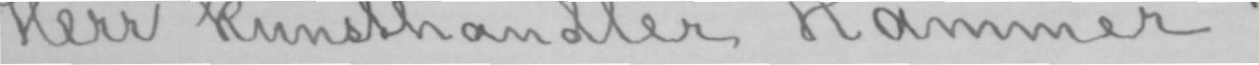Herr kunsthandler Hammer!
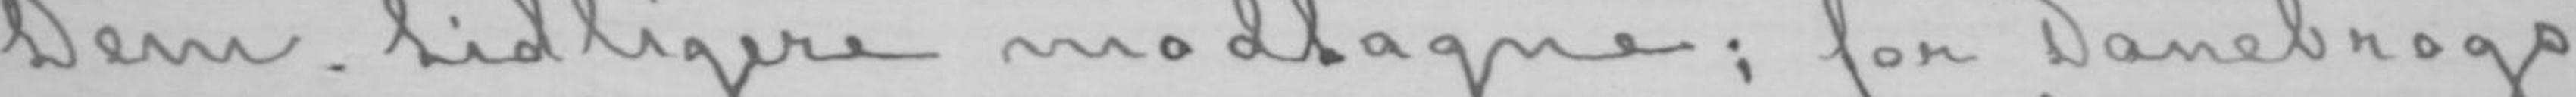Dem tidligere modtagne; for Danebrogs-
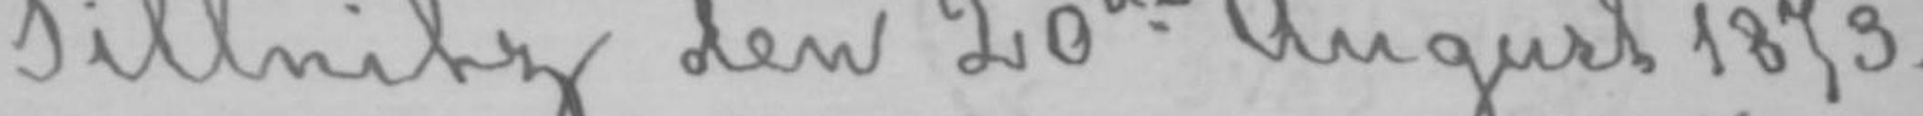Pillnitz den 20de August 1873.
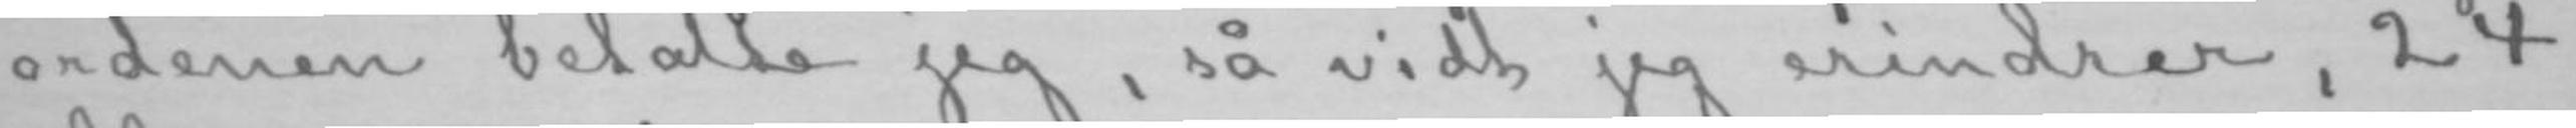ordenen betalte jeg, så vidt jeg erindrer, 24
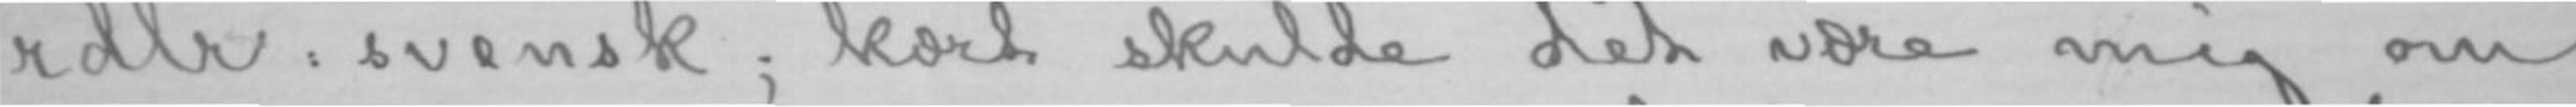rdlr: svensk; kært skulde det være mig om
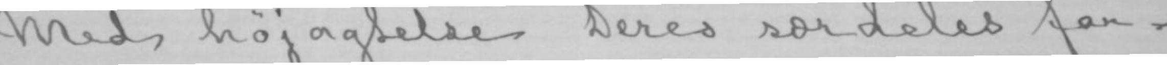Med højagtelse Deres særdeles for-
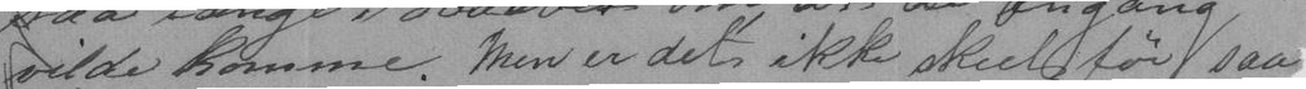vilde komme. Men er det ikke skeet før, saa
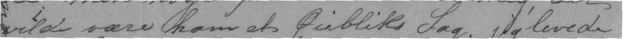vilde være ham et Øiebliks Sag; jeg levede
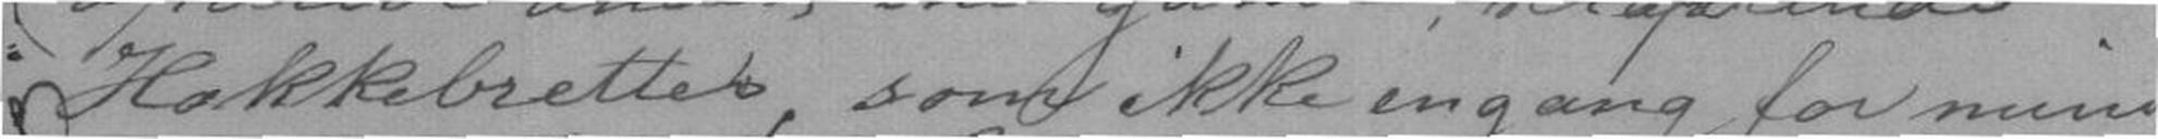Hakkebrettes, som ikke engang for mine
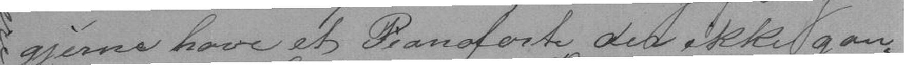gjerne have et Pianoforte der ikke gan-
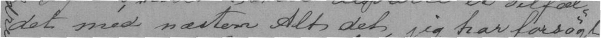det med næsten Alt det, jeg har forsøgt
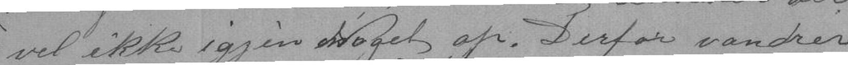vel ikke igjen Noget op. Derfor vandrer
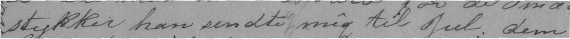stykker han sendte mig til Jul; dem
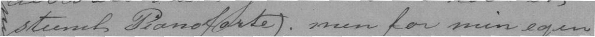stumt Pianoforte); men for min egen
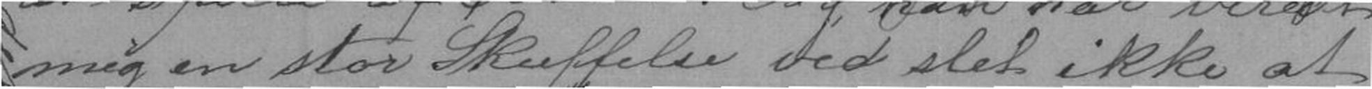mig en stor Skuffelse ved slet ikke at
